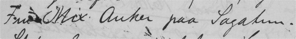Fru  Mix Anker paa Sagatun.
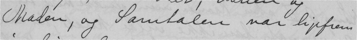Maden, og Samtalen var ligefrem
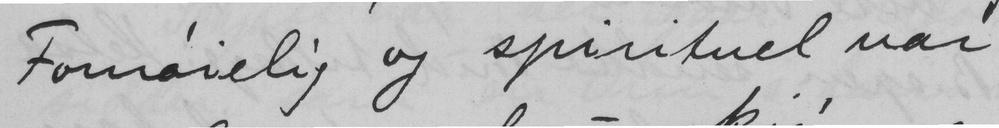Fornøielig og spirituel var
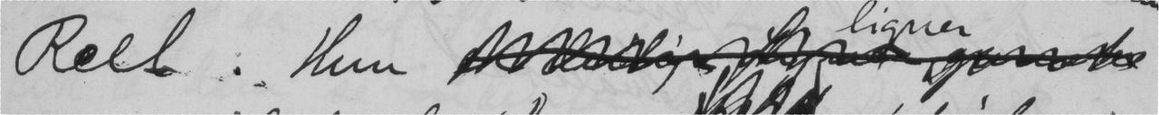reel. Hun
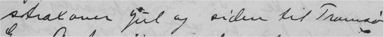strax over Jul og siden til Tromsø
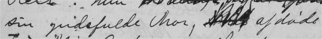sin yndefulle Mor,  af døds
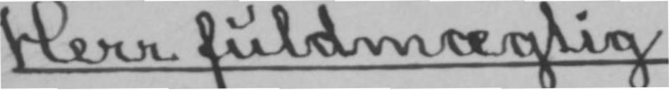Herr fuldmægtig
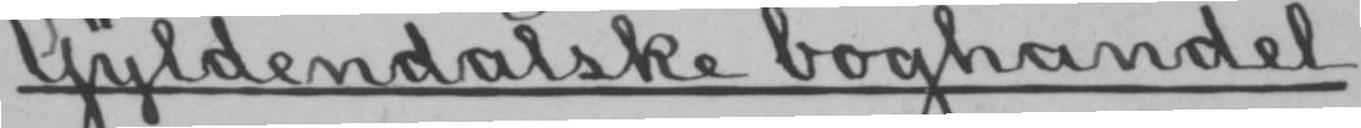Gyldendalske boghandel.
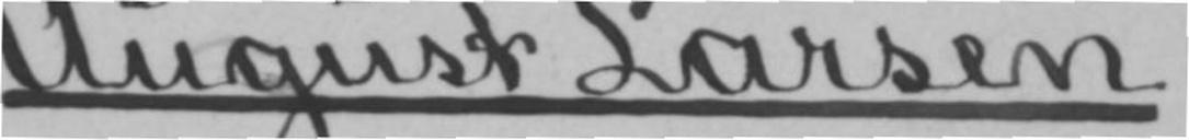August Larsen.
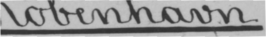København.
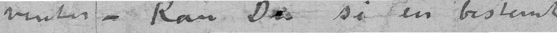venter – Kan Du si en bestemt
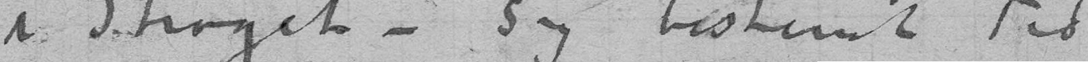i Strøget – Sig bestemt Tid
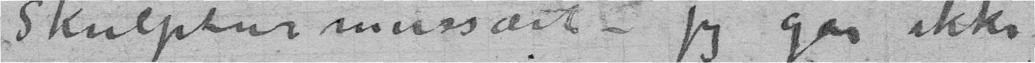Skulpturmusæet – Jeg går ikke
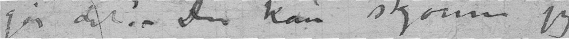går det? – Du kan skjønne jeg
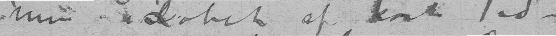men i løbet af kort Tid –
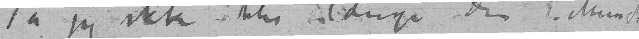da jeg ikke blir længe Din E. Munch
